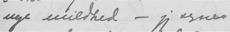nye mildhed - jeg synes
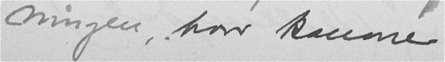ningen, hvor kanoner
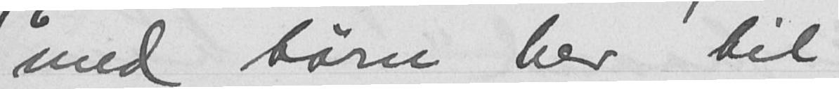med børn her til
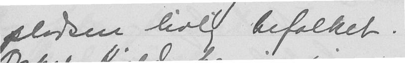pladsen livlig befolket.
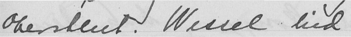oberstlnt. Wessel hid
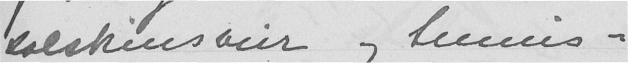solskinsveir og tennis-
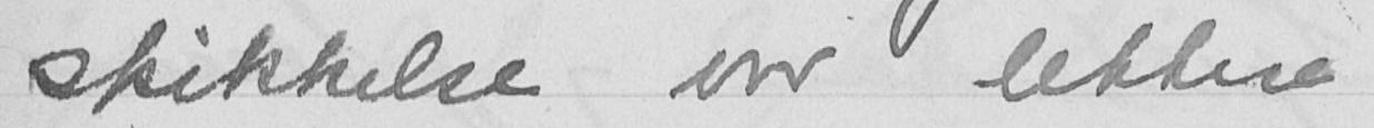skikkelse var lettere
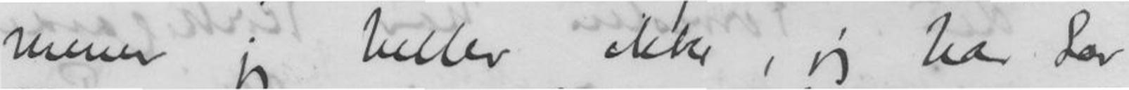mener jeg heller ikke, jeg har Lov
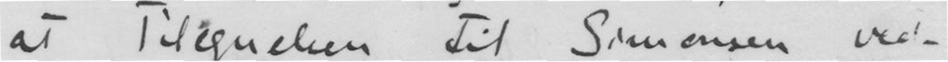at Tilegnelsen til Simonsen ved-
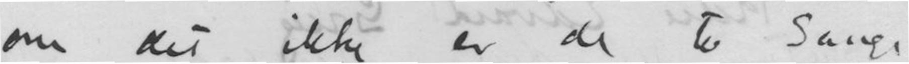om det ikke er de to Sange,
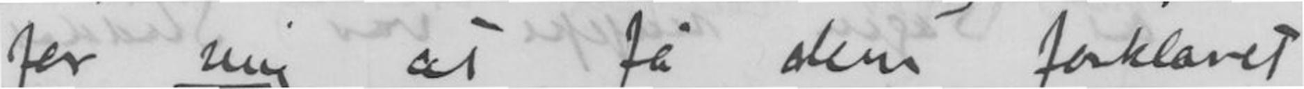for mig at få dem forklaret
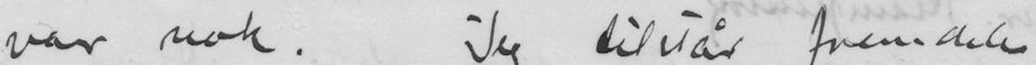var nok. Jeg tilstår fremdeles
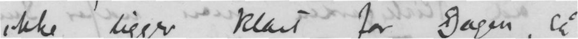ikke ligger klart for Dagen, så
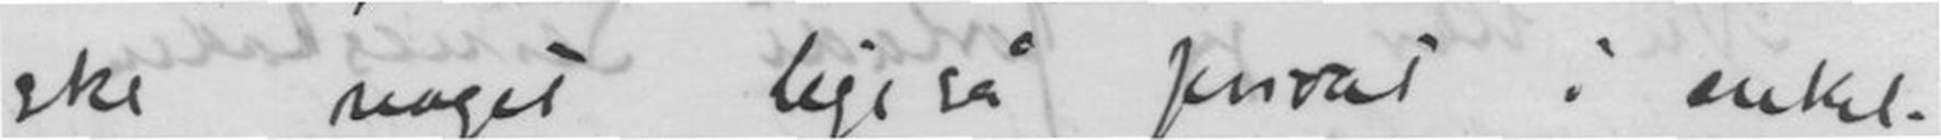ske noget ligeså privat i enkel-
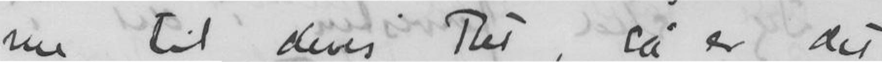me til deres Ret, så er det
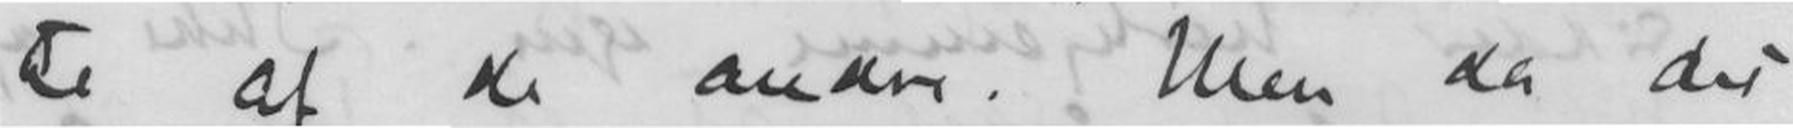te af de andre. Men da det
